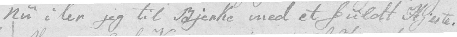nu iler jeg til Bjerke med et fuldt Hjerte.
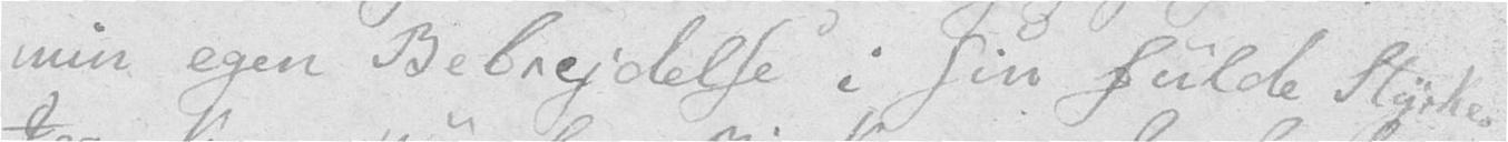min egen Bebrejdelse i sin fulde Styrke.
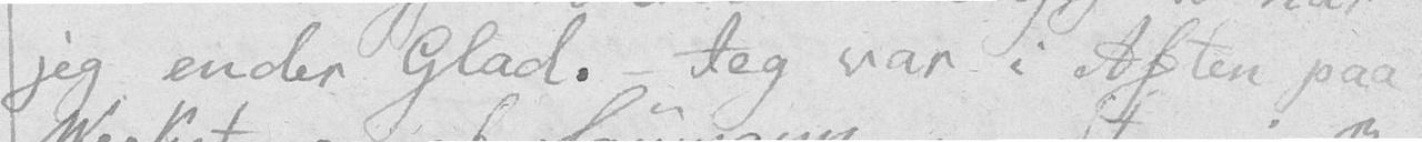jeg ender Glad. – Jeg var i Aften paa
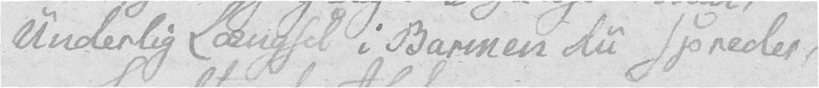Underlig Længsel i Barmen du spreder,
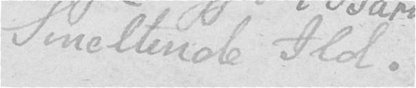Smeltende Ild.
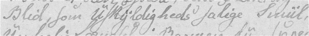Blid, som Uskyldigheds salige Smiil,
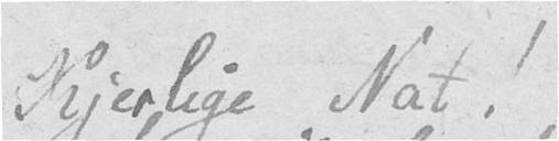Kjerlige Nat!
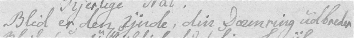Blid er den ynde, din Dæmring udbreder
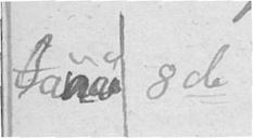Janar 8de
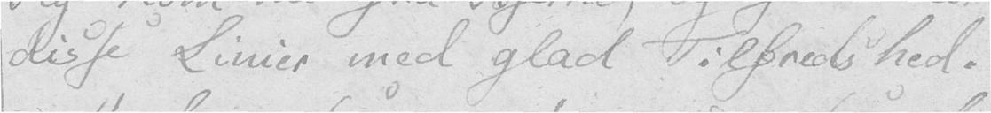disse Linier med glad Tilfredshed.
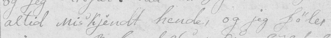altid Miskjendt hende, og jeg føler
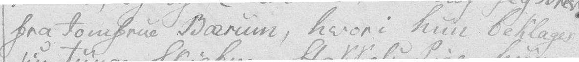fra Jomfrue Bærum, hvori hun beklager
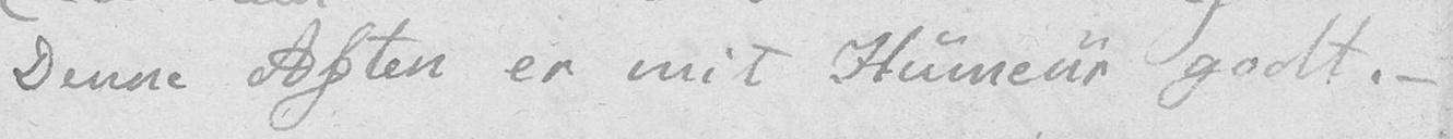Denne Aften er mit Humeur godt. –
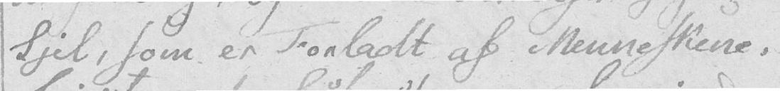Sjel, som er Forladt af Menneskene.
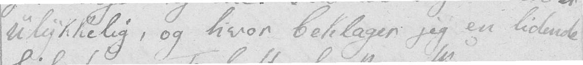ulykkelig, og hvor beklager jeg en lidende
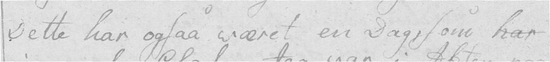Dette har ogsaa været en Dag, som har
